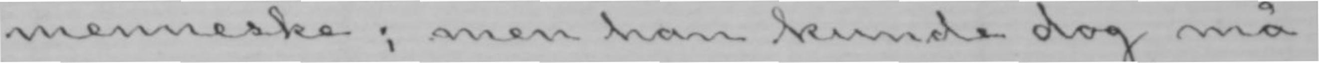menneske; men han kunde dog må-
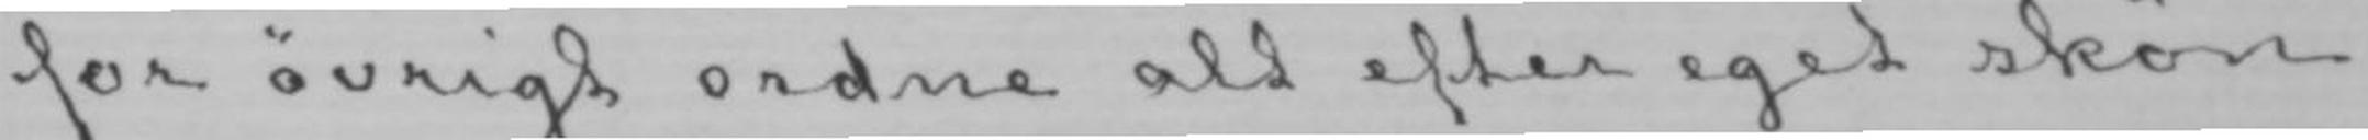for øvrigt ordne alt efter eget skøn.
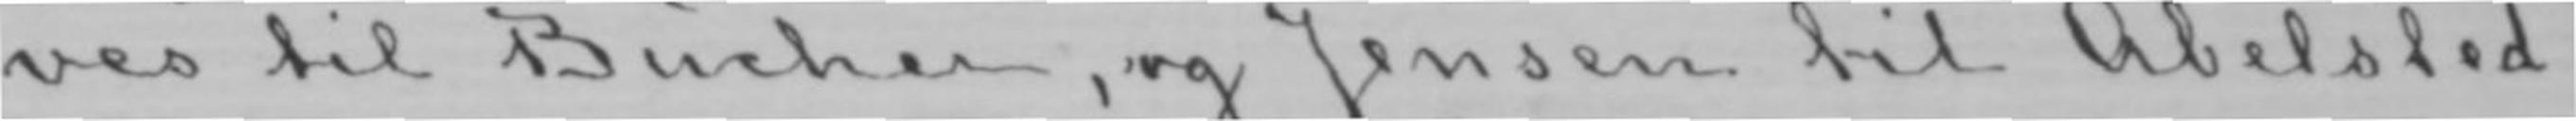ves til Bucher, og Jensen til Abelsted,
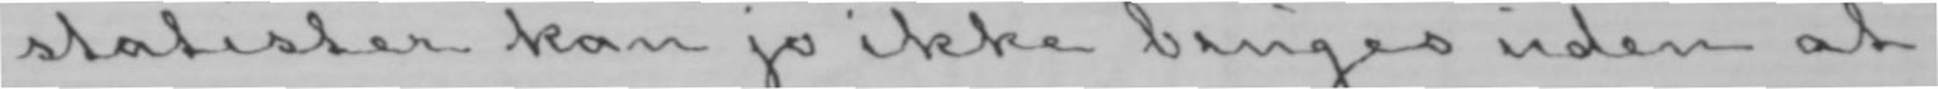statister kan jo ikke bruges uden at
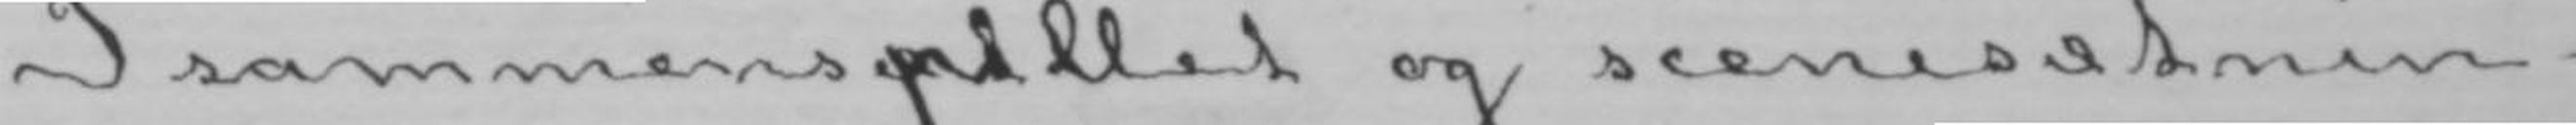I sammenspillet og scenesætnin-
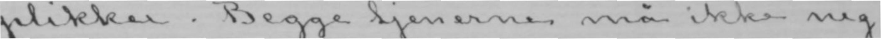plikker. Begge tjenerne må ikke neg-
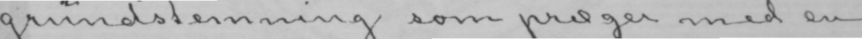grundstemning som præger med en
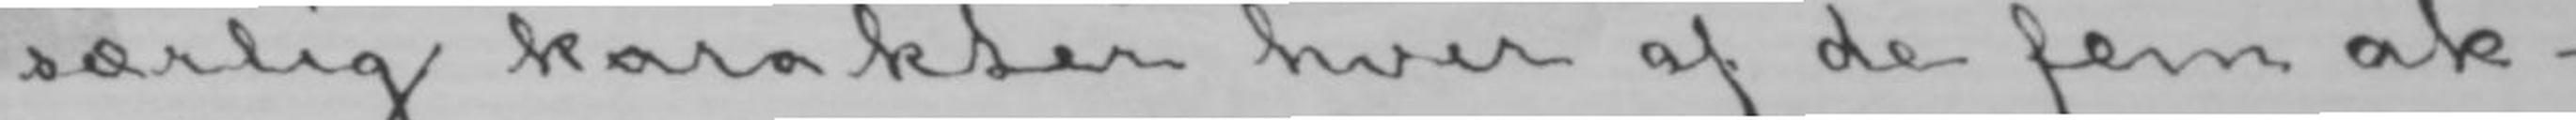særlig karakter hver af de fem ak-
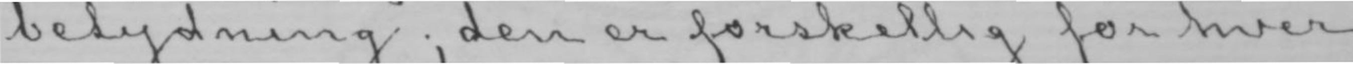betydning; den er forskellig for hver
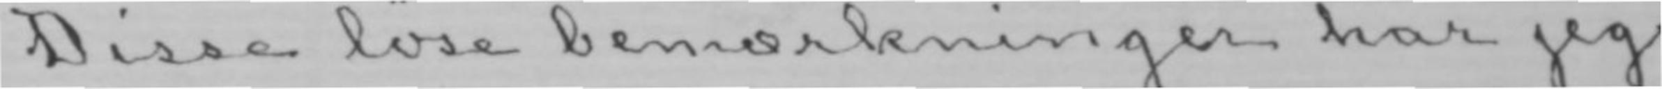Disse løse bemærkninger har jeg
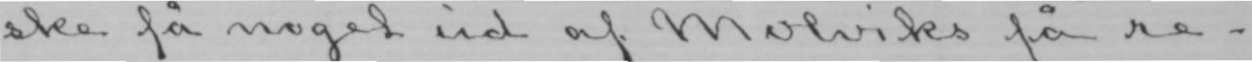ske få noget ud af Molviks få re-
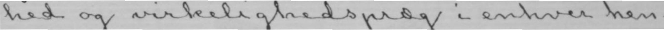hed og virkelighedspræg i enhver hen-
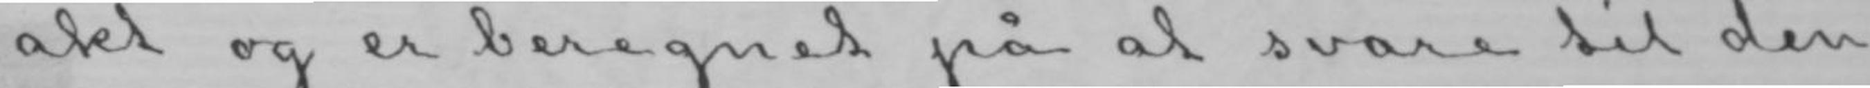akt og er beregnet på at svare til den
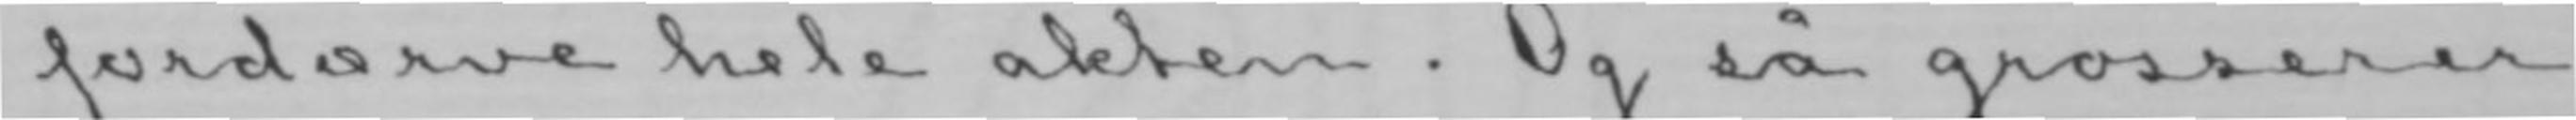fordærve hele akten. Og så grosserer
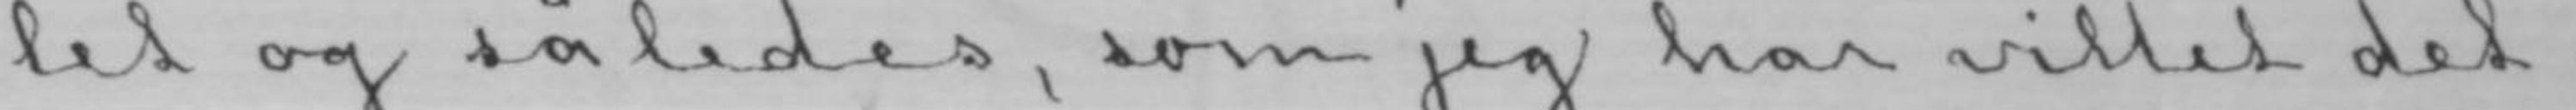let og således, som jeg har villet det.
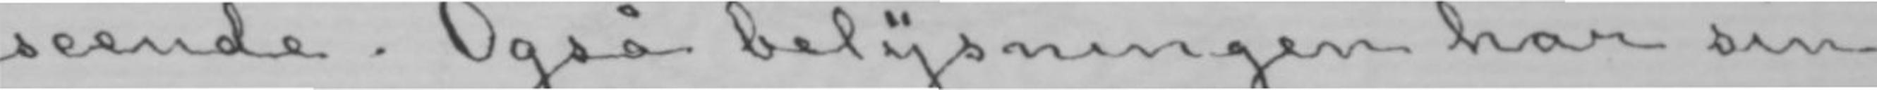seende. Også belysningen har sin
